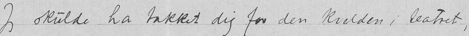Jeg skulde ha takket dig for den kvelden i teatret,
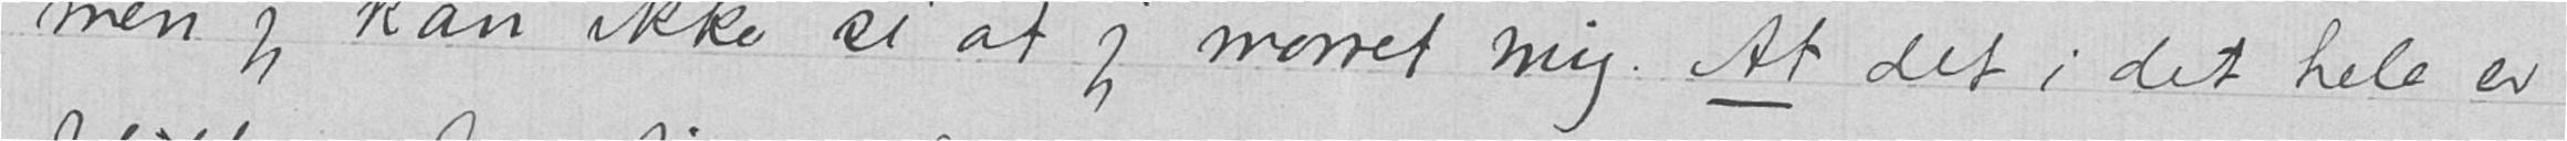men jeg kan ikke si at jeg morret mig. At det i det hele er
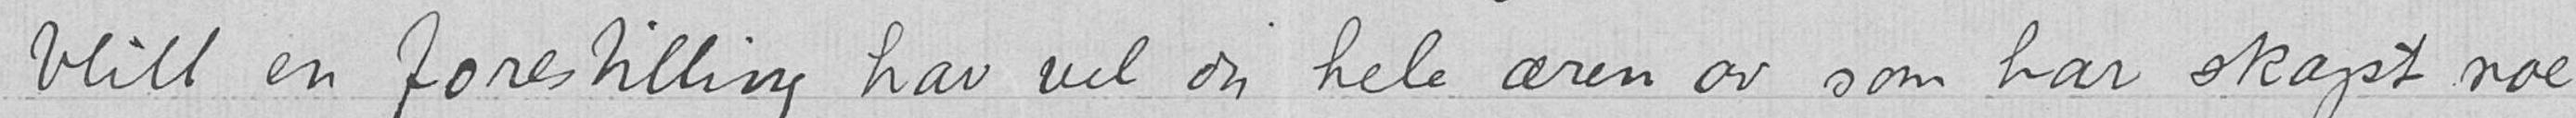blitt en forestilling har vel du hele æren av som har skapt noe
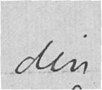din
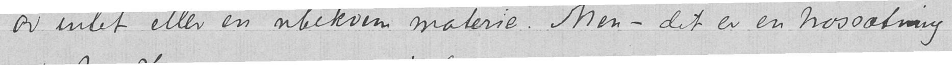av intet eller en ubekvem materie. Men - det er en trossætning
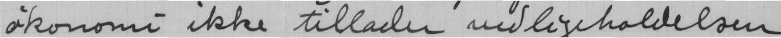økonomi ikke tillader vedligeholdelsen
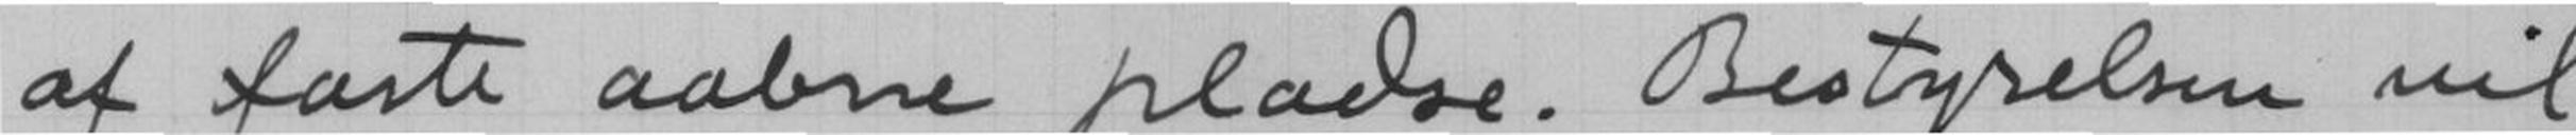af faste aabne pladse. Bestyrelsen vil
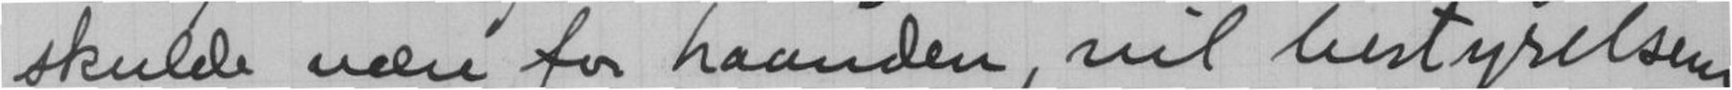skulde være for haanden, vil bestyrelsens
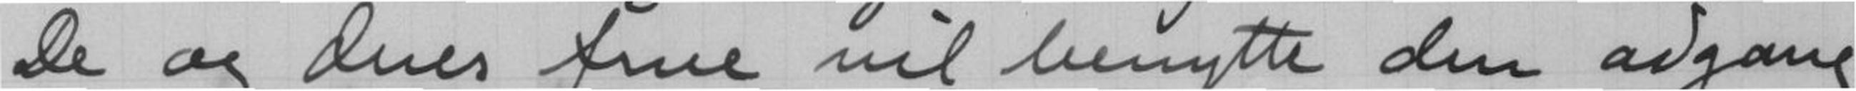De og Deres frue vil benytte den adgang
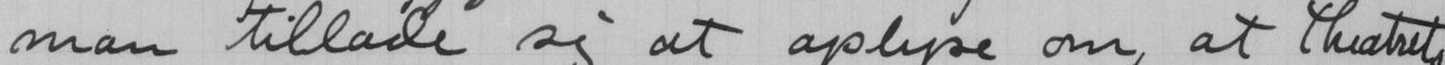man tillade sig at oplyse om, at theatrets
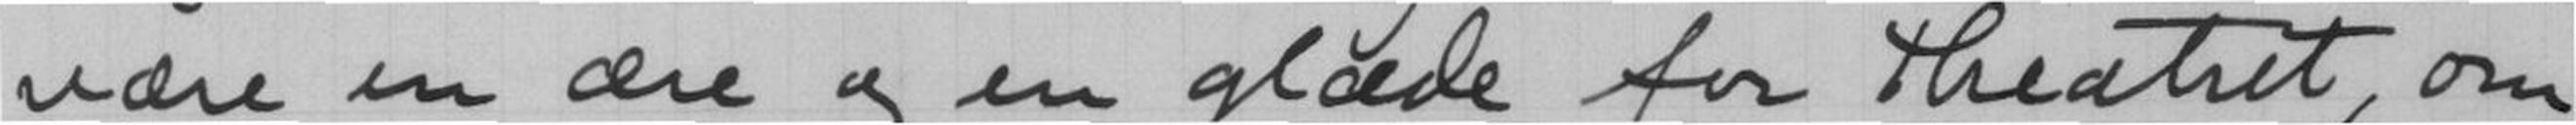være en ære og en glæde for Theatret, om
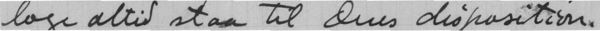loge altid staa til Deres disposition.
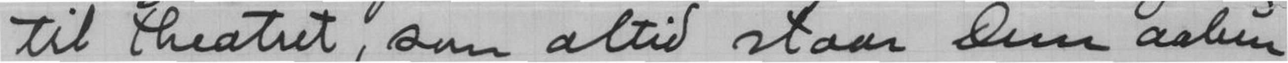til theatret, som altid staar Dem aaben
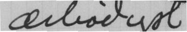ærbødigst
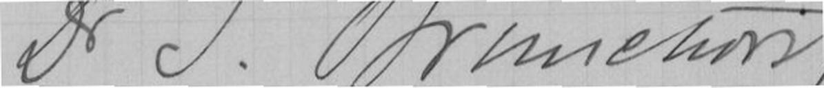Dr I. Brunchorst
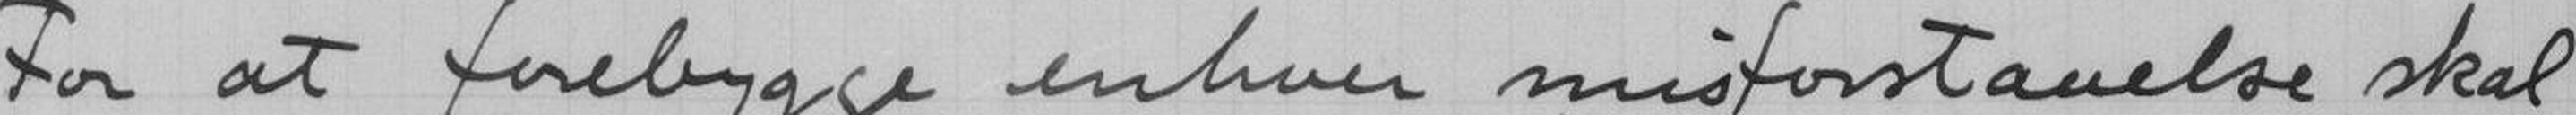For at forebygge enhver misforstaaelse skal
